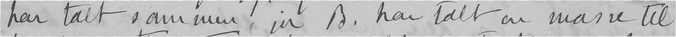har talt sammen, ja B. har talt en masse til
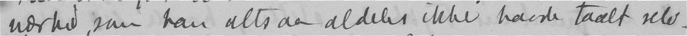nærhed, som han altsaa aldeles ikke havde taalt selv.
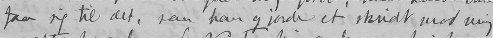faa sig til det, saa han gjorde et skridt mod mig
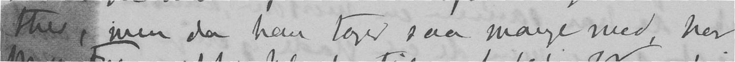the, men da han tager saa mange med, har
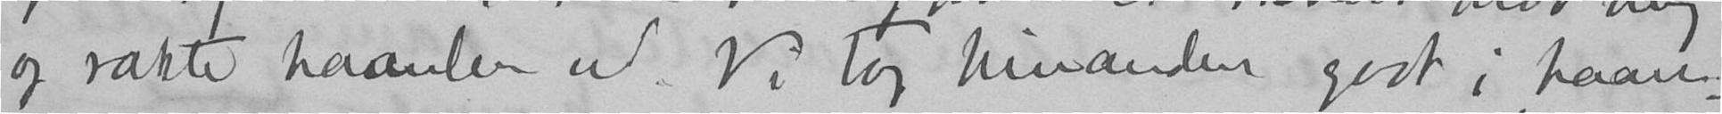og rakte haanden ud. Vi tog hinanden godt i haan-
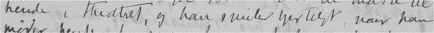hende i theatret, og han smiler hjerteligt, naar han
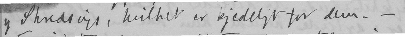og Skredsvigs, hvilket er kjedeligt for dem. -
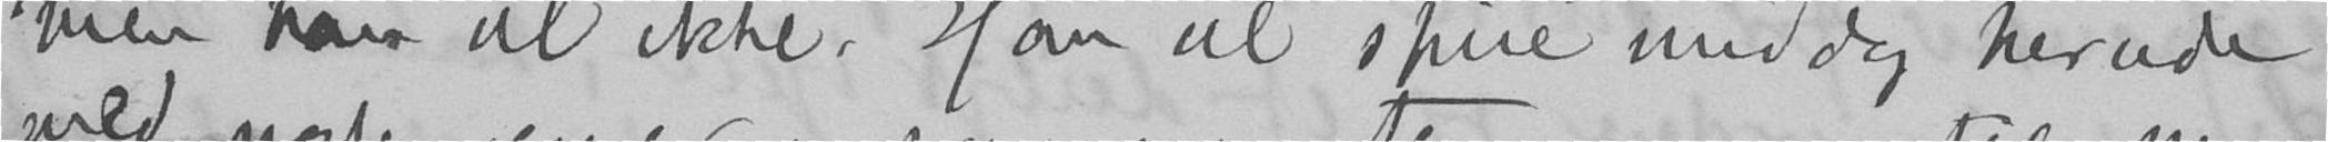men han vil ikke. Han vil spise middag herude
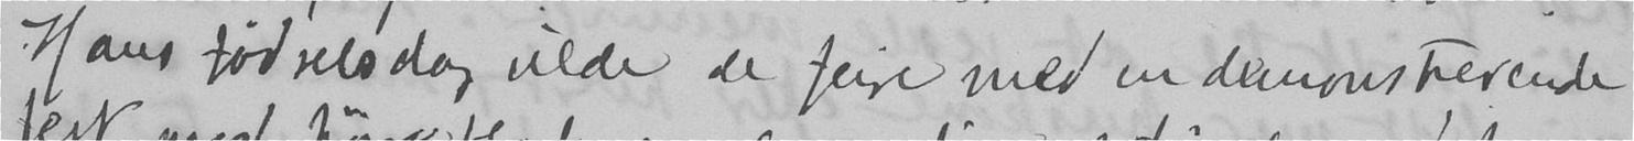Hans fødselsdag vilde de feire med en demonstrerende
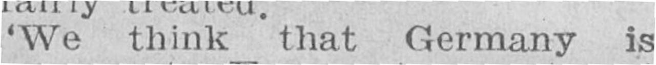We think that Germany is
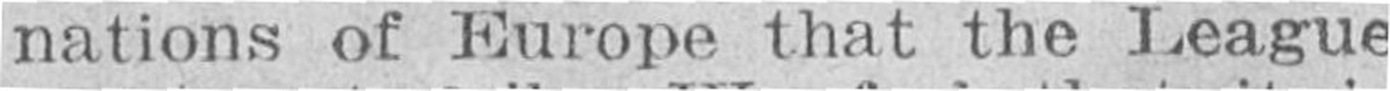nations of Europe that the League
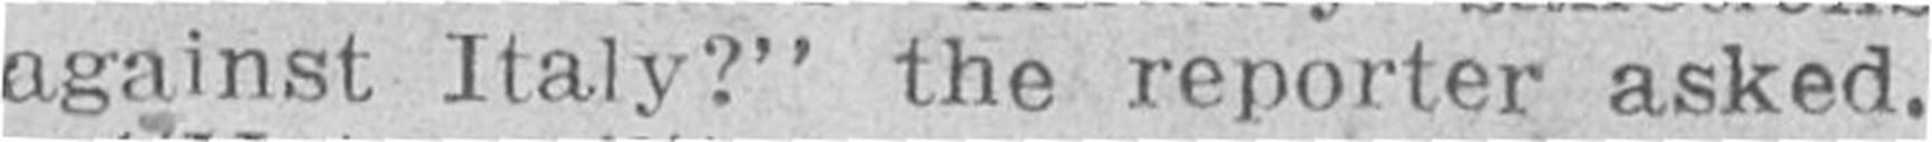against Italy?" the reporter asked.
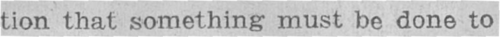tion that something must be done to
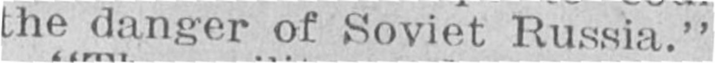the danger of Soviet Russia."
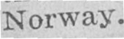Norway.
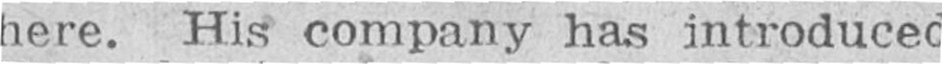here. His company has introduced
